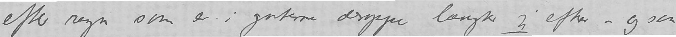efter regn som er i gaterne deroppe længter jeg efter – og saa den
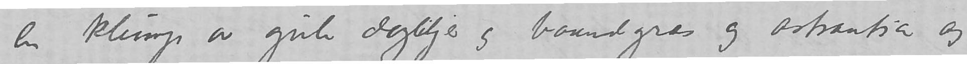en klump av gule dagliljer og baandgræs og astrantia og
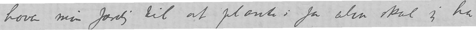haven min færdig til at plante i for alvor skal jeg ha
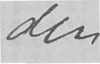din
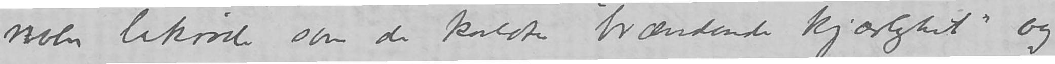noen lakrøde som de kalder «brændende kjærlighet» og
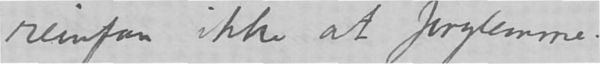reinfan ikke at forglemme.
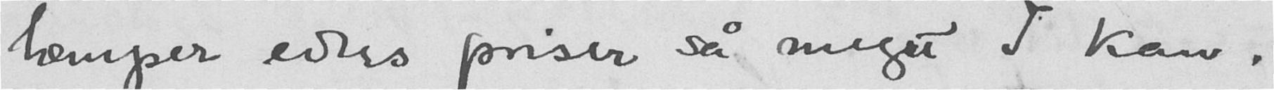læmper eders priser så meget I kan.
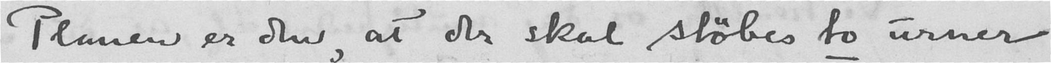Planen er den, at der skal støbes to urner
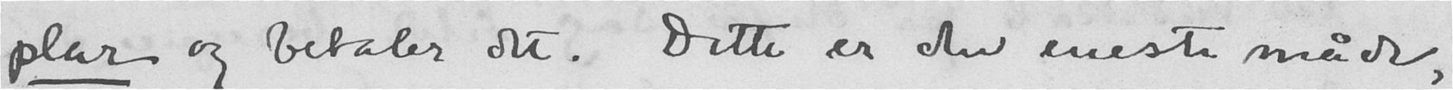plar og betaler det. Dette er den eneste måde,
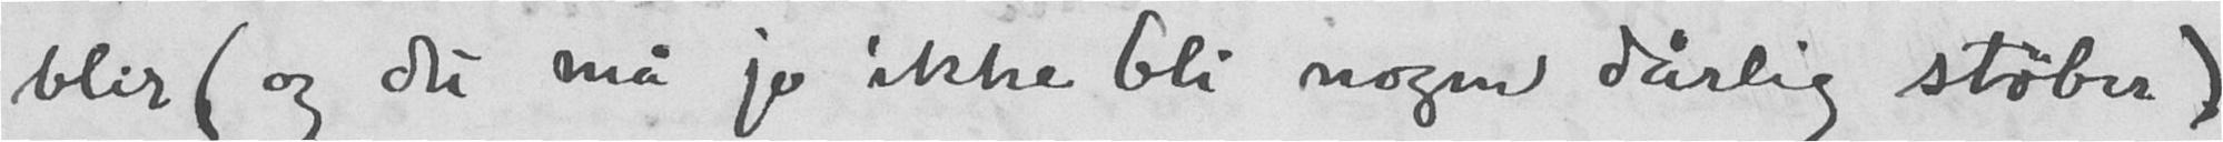blir (og det må jo ikke bli nogen dårlig støber)
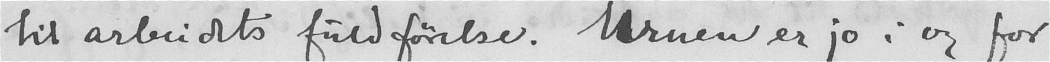til arbeidets fuldførelse. Urnen er jo i og for
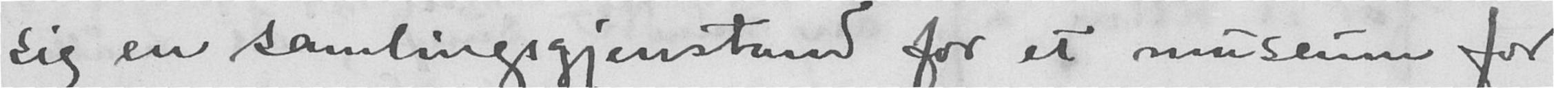sig en samlingsgjenstand for et museum for
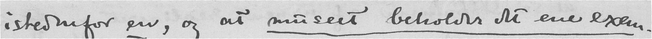istedenfor en, og at museet beholder det ene exam-
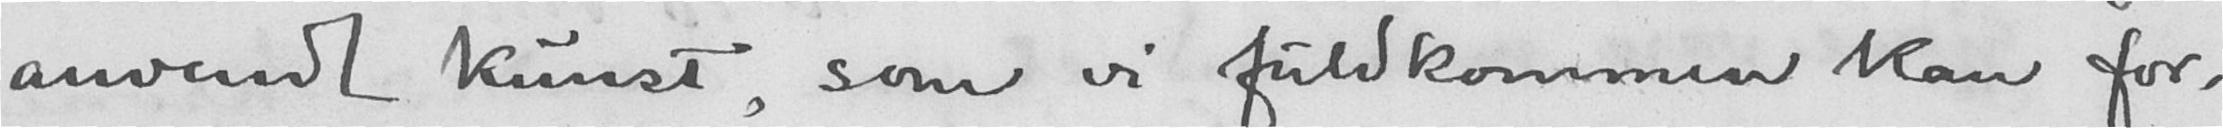anvendt kunst, som vi fuldkommen kan for-
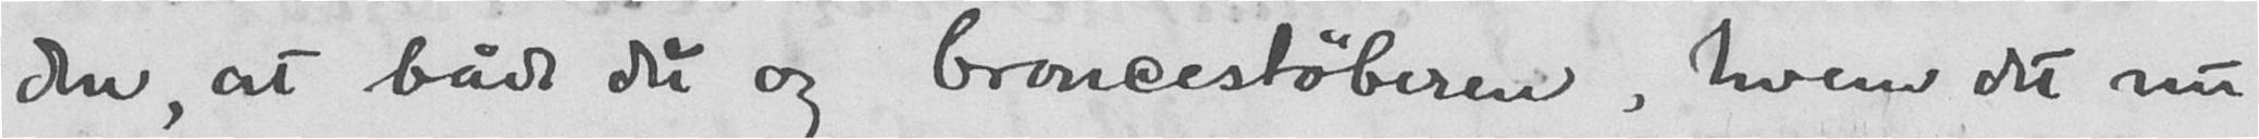den, at både du og broncestøberen, hvem det nu
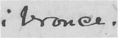i bronce.
